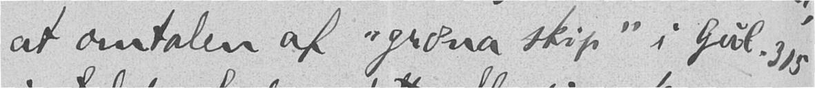at omtalen af "græna skip" i Gul. 315
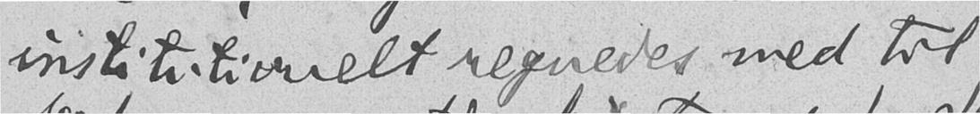institutionelt regnedes med til
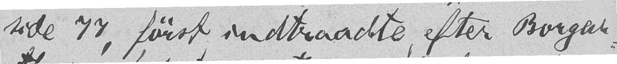side 77, først indtraadte efter Borgar-
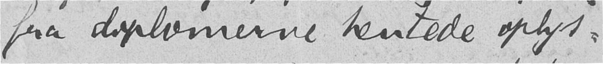fra diplomerne hentede oplys-
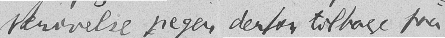skrivelse peger derfor tilbage paa
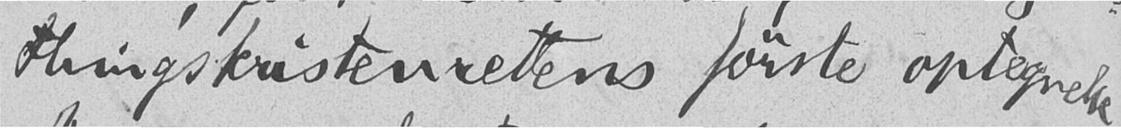thingskristenrettens første optegnelse.
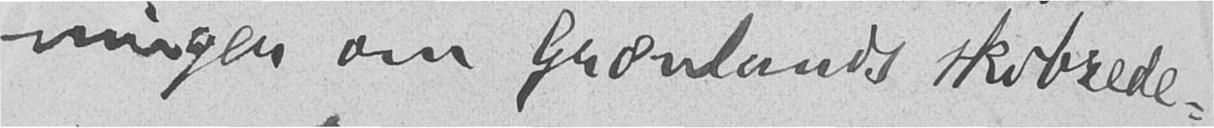ninger om Grønlands skibrede-
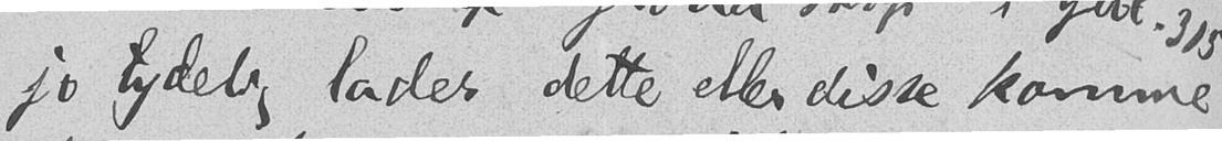jo tydelig lader dette eller disse komme
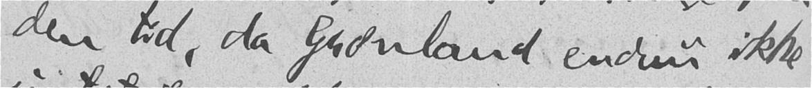den tid, da Grønland endnu ikke
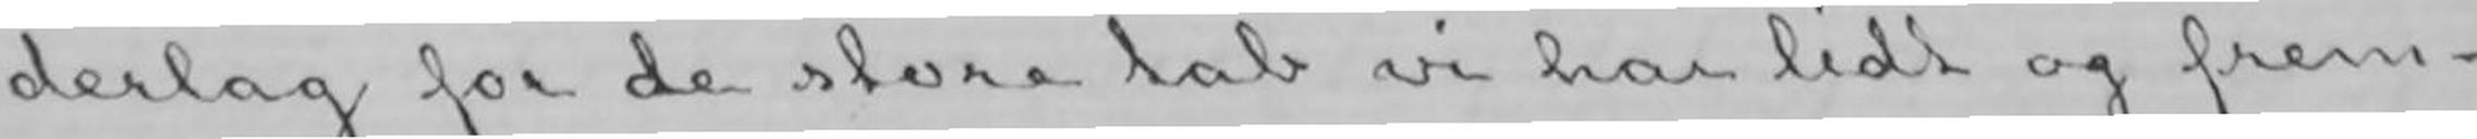derlag for de store tab vi har lidt og frem-
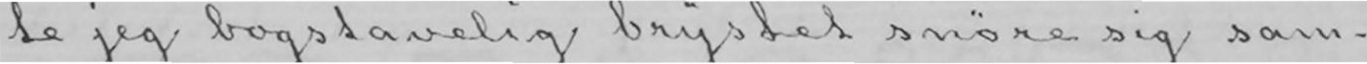te jeg bogstavelig brystet snøre sig sam-
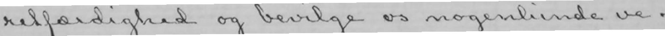retfærdighed og bevilge os nogenlunde ve-
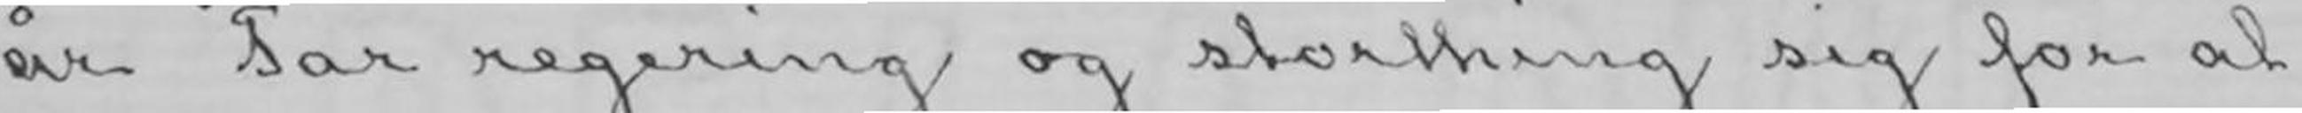år. Tar regering og storthing sig for at
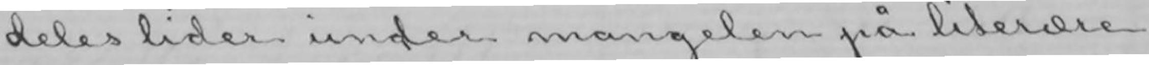deles lider under mangelen på literære
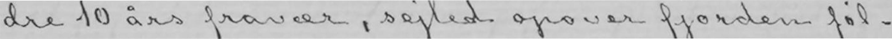dre 10 års fravær, sejled opover fjorden føl-
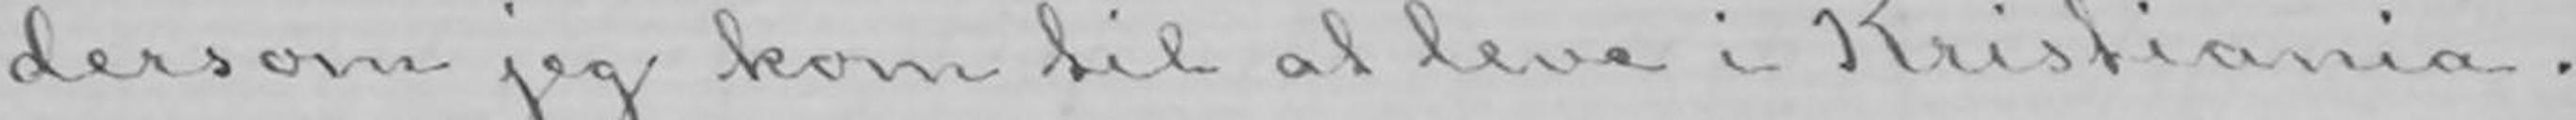dersom jeg kom til at leve i Kristiania.
